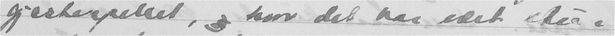gjestespillet, og hvor det har vært idig-
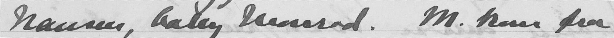Nansen, Cally Monrad. M. kom fra
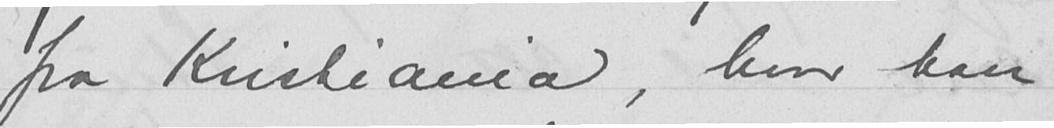fra Kristiania, hvor han
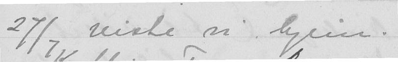27/7 reiste vi hjem.
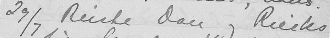29/7 Reiste Don og Riecks
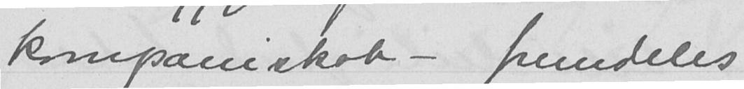kompaniskab - fremdeles
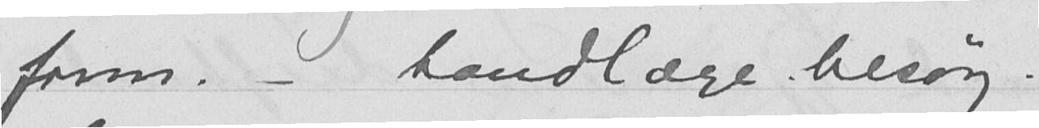form. - tandlægebesøg.
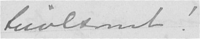tvivlsomt!
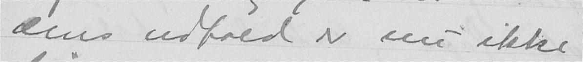dens udfald er nu ikke
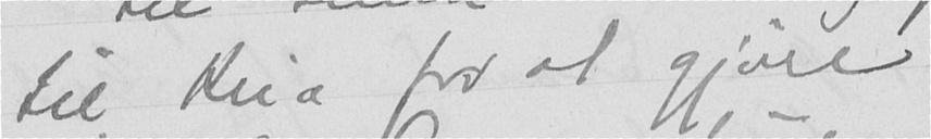til Kria for at gjøre
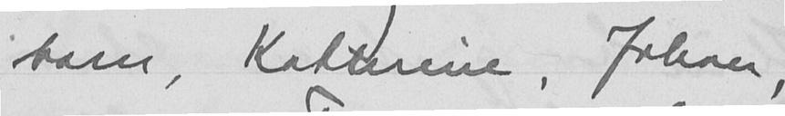barn, Kathrine, Johan,
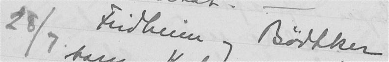28/7 Fridheim og Bødtker
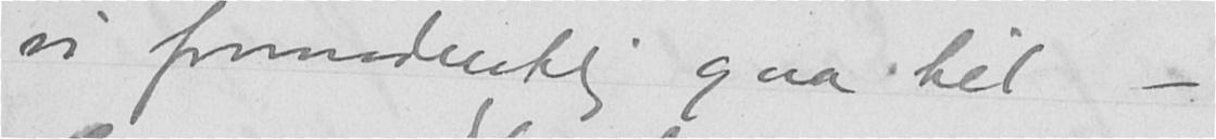vi formodentlig gaa til -
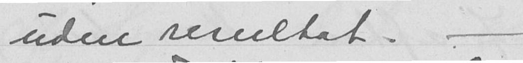uden resultat. -
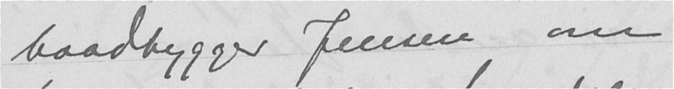baadbygger Jensen om
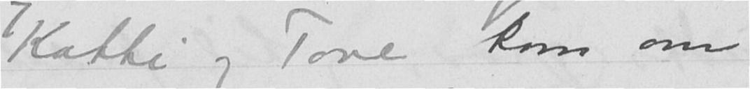Katti og Tove kom om
